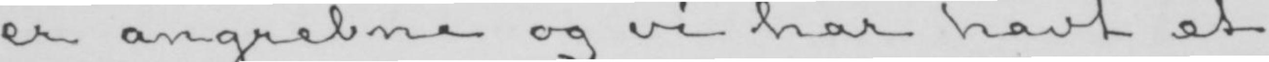er angrebne og vi har havt et
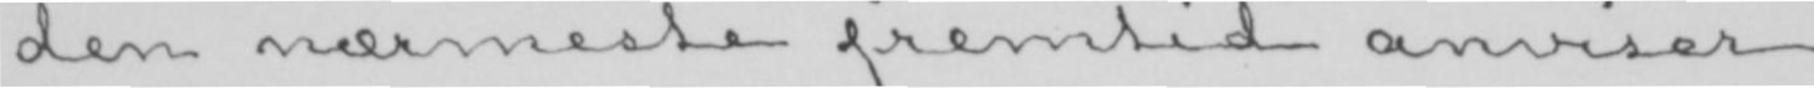den nærmeste fremtid anviser
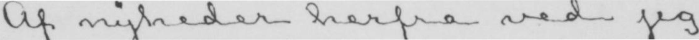Af nyheder herfra ved jeg
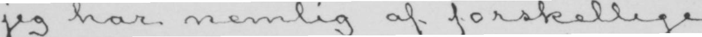jeg har nemlig af forskellige
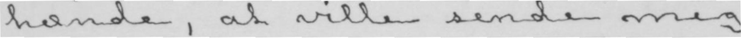hænde, at ville sende mig
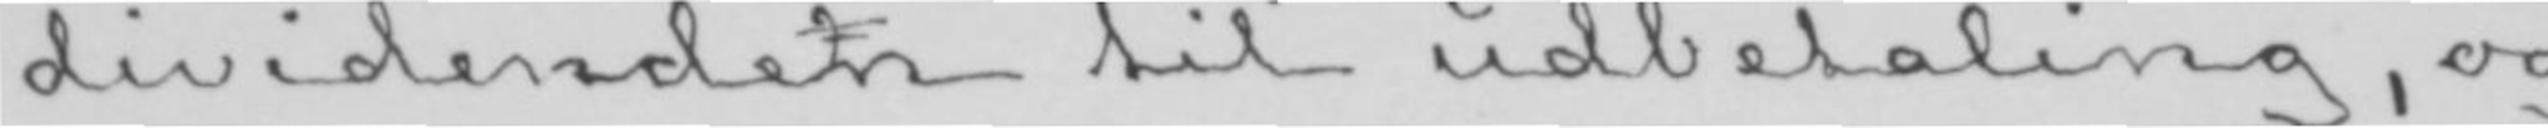dividenden til udbetaling, og
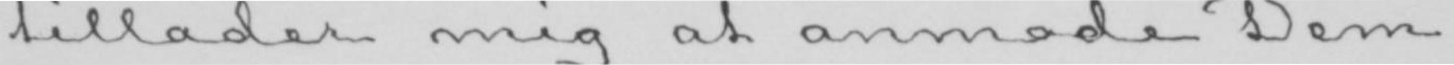tillader mig at anmode Dem
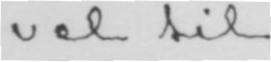vel til.
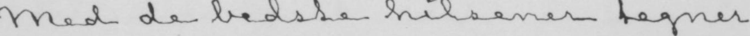Med de bedste hilsener tegner
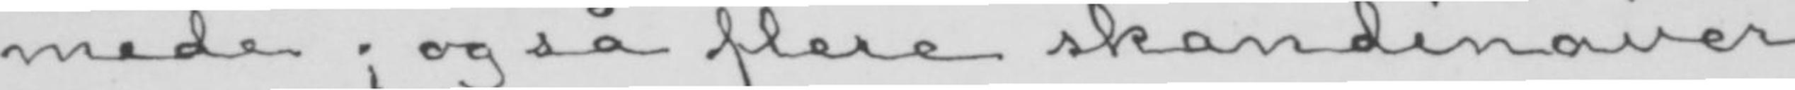mede; også flere skandinaver
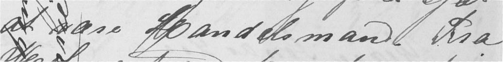at være Handelsmand. Fra
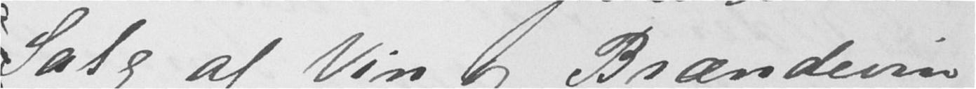Salg af Vin og Brændevin
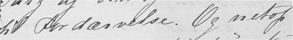til Fordærvelse. Og netop
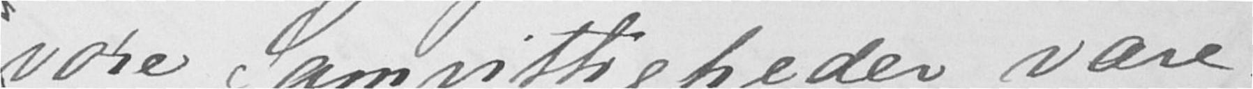vore Samvittigheder være.
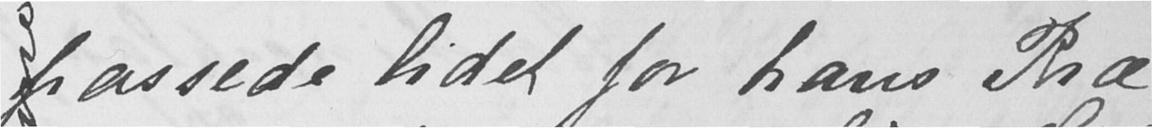passede lidet for hans Præ-
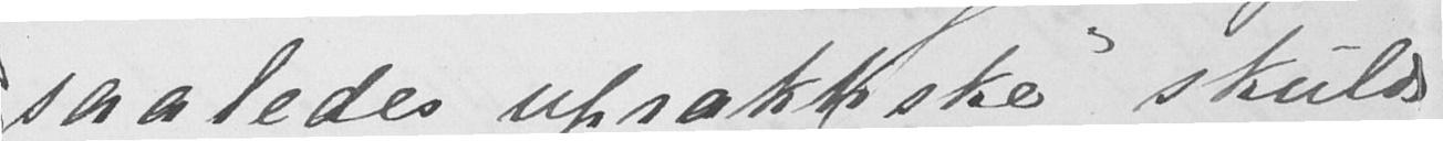saaledes "upraktiske" skulde
